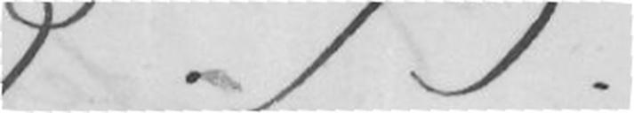B. B.
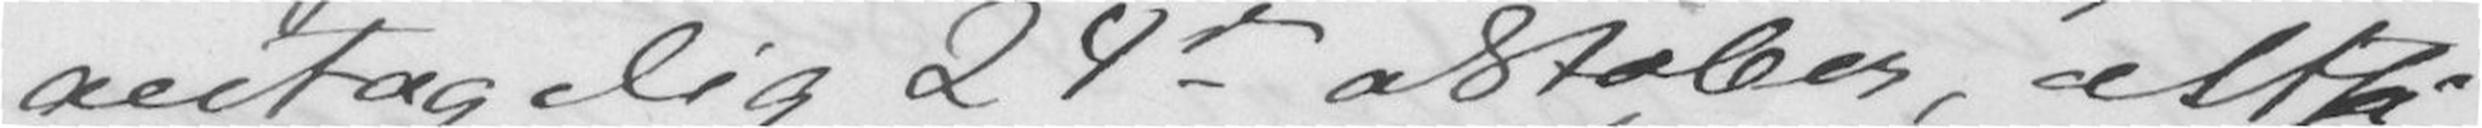antagelig 24de oktober, altså
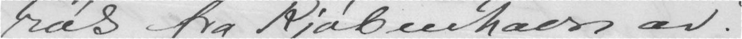røk fra Kjøbenhavn av?
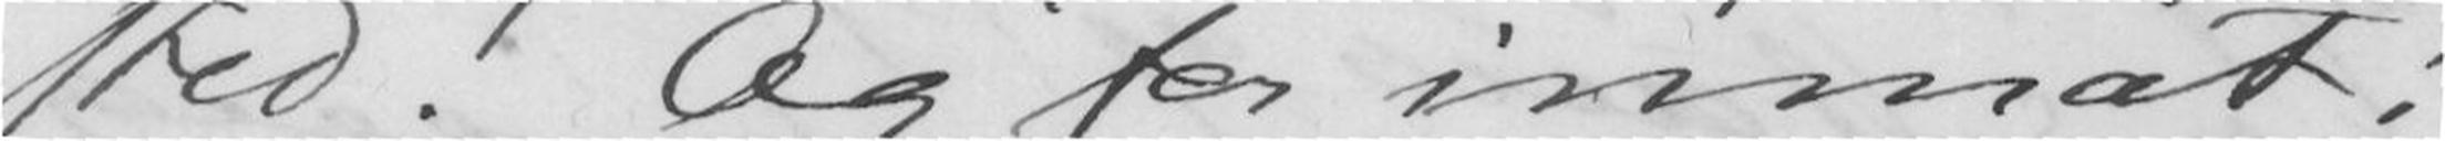sted! Og for inmat!
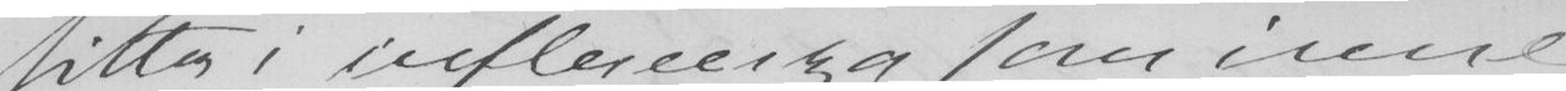sitter i influenza som inne
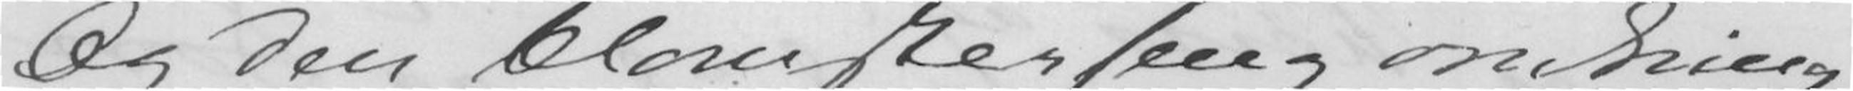Og den blomsterseng omkring
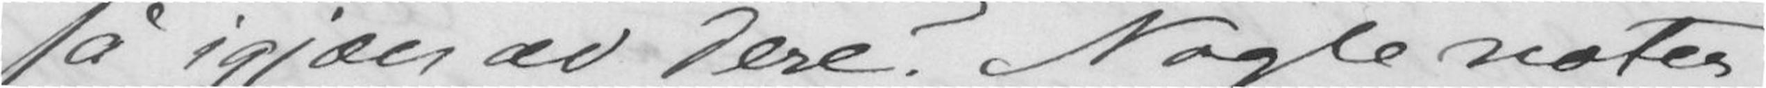så igjæn av dere? Nogle noter
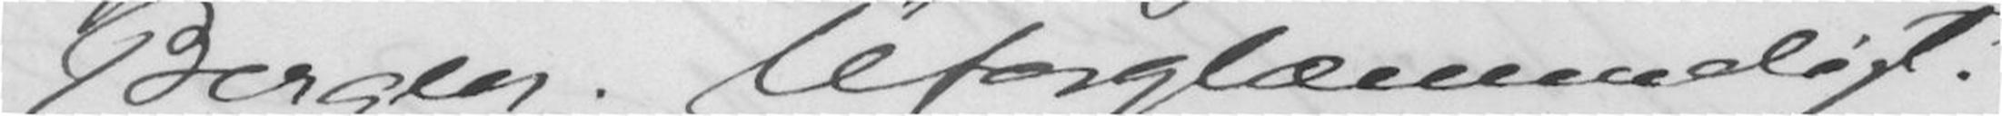i Bergen. Uforglæmmeligt.
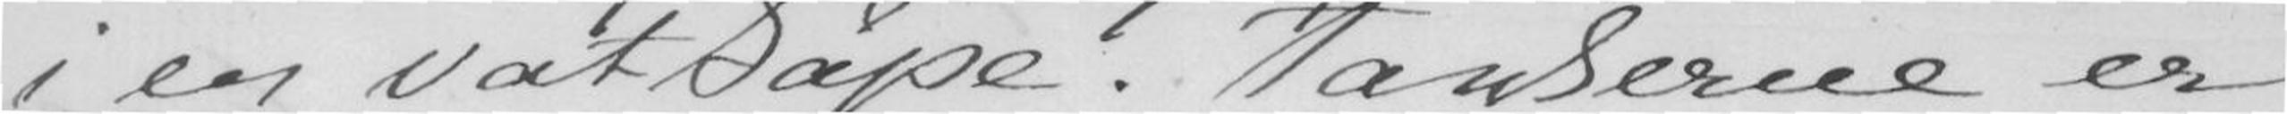i en vatkåpe. Tankerne er
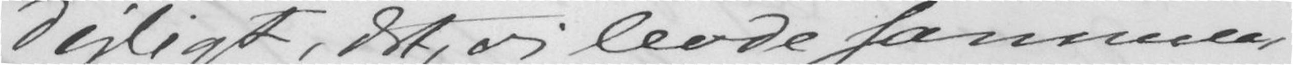dejligt, det, vi levde sammen
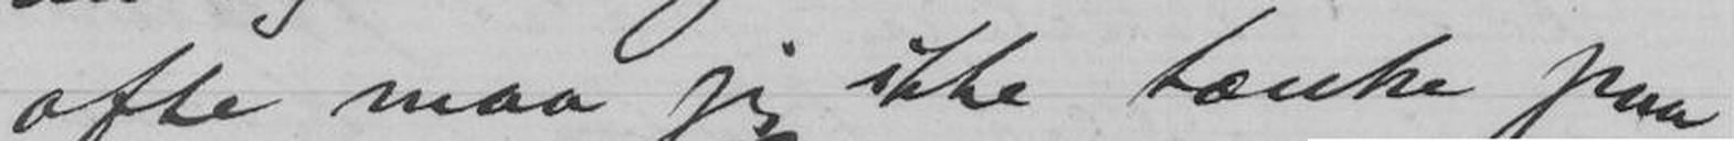ofte maa jeg ikke tænke paa
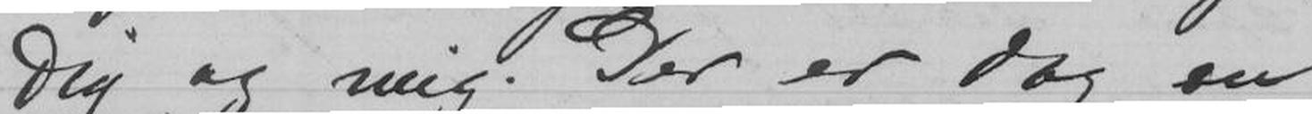Dig og mig. Der er dog en
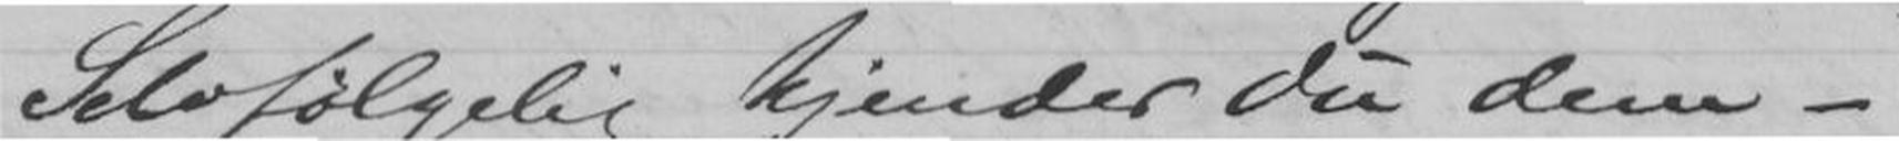Selvfølgelig kjender Du dem -
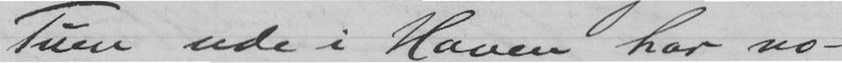Tuen ude i Haven har no-
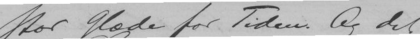stor Glæde for Tiden. Og det
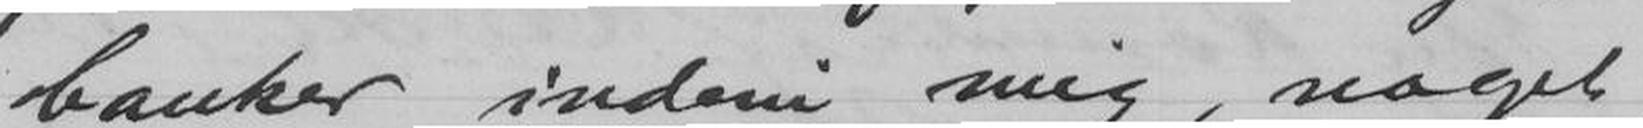banker indeni mig, noget,
